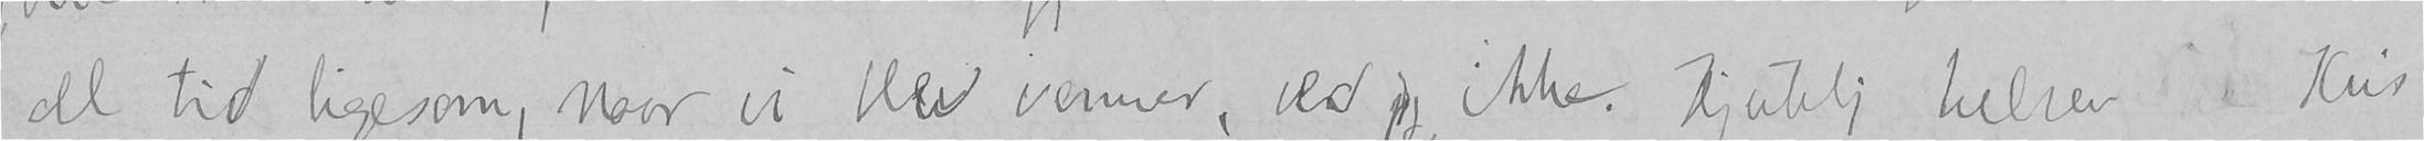al tid ligesom, naar vi blev venner, ved jeg ikke. Hjertelig hilsen til Kris
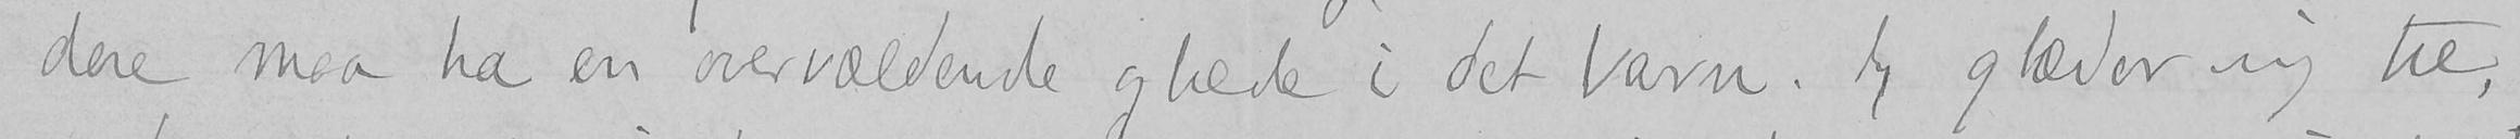dere maa ha en overvældende glæde i det barn. Jeg glæder mig til,
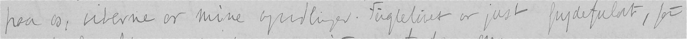paa os, viberne er mine yndlinger. Fuglelivet er just frydefuldt, for
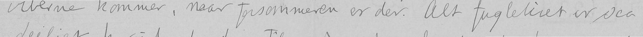viberne kommer, naar forsommeren er der. Alt fuglelivet er saa
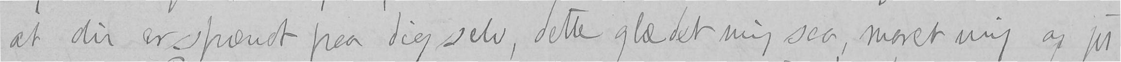at du er spændt paa dig selv, dette glædet mig saa, moret mig og jeg
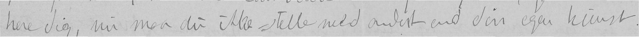here dig, nu maa du ikke stelle med andet end din egen kunst.
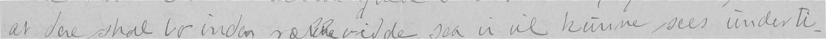at dere skal bo inden rækkevidde, saa vi vil kunne sees underti-
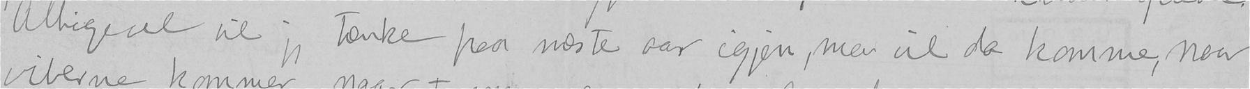Alligevel vil jeg tænke paa næste aar igjen, men vil da komme, naar
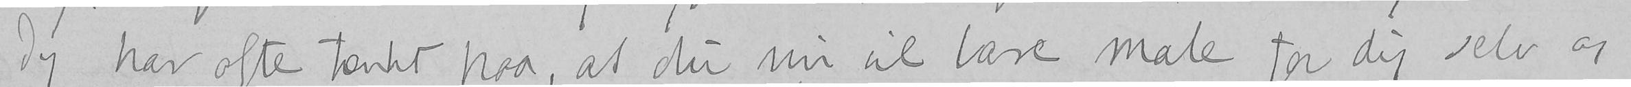Jeg har ofte tænkt paa, at du nu vil bare male for dig selv og
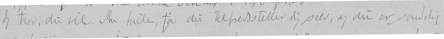Jeg tror, du vil ikke hvile, for du tilfredsstiller dig selv, og du er sandelig
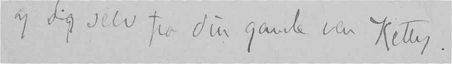og dig selv fra din gamle ven Kitty.
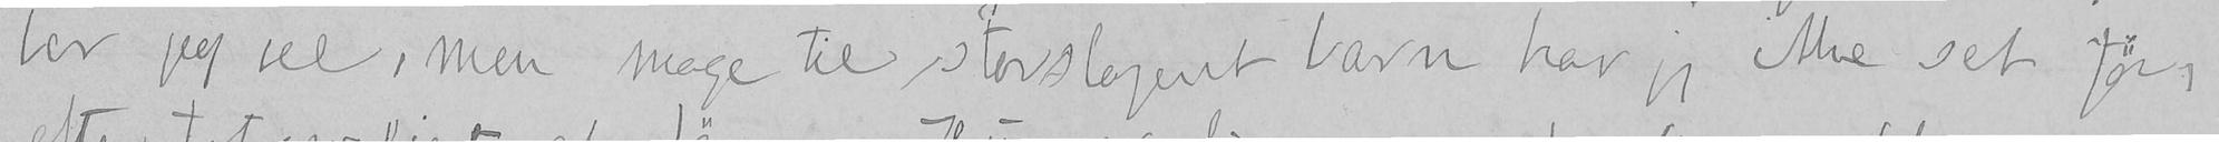ber jeg vel, men mage til storslagent barn har jeg ikke set før,
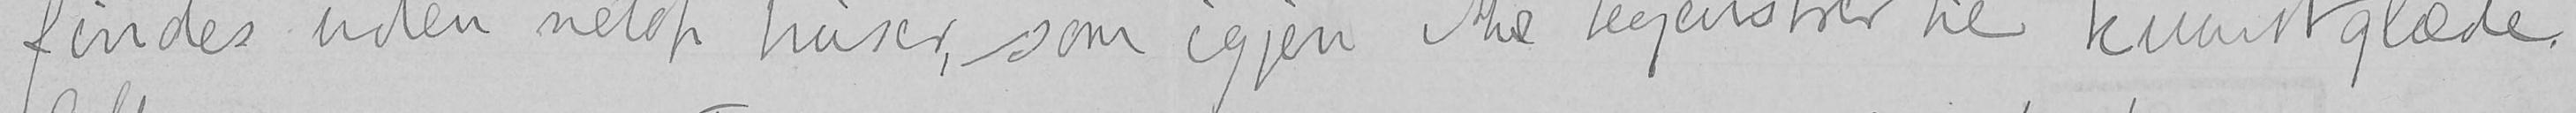findes uden netop huser, som igjen ikke begeistrer til kunstglæde.
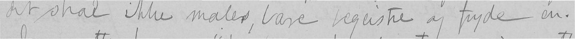det skal ikke males, bare begeistre og fryde en.
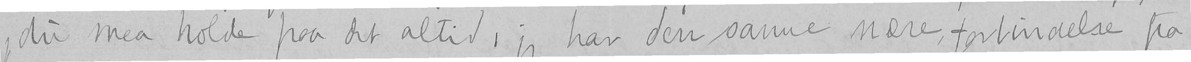du maa holde paa det altid, jeg har den sanne nære, forbindelse fra
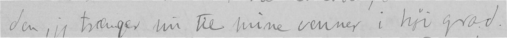den, jeg trænger nu til mine venner i høi grad.
